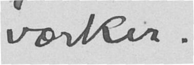værker.
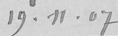19.11.07
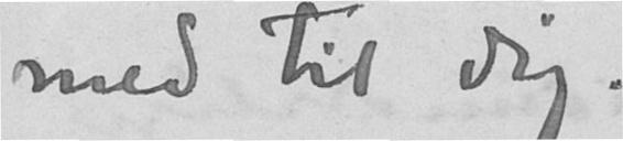med til dig.
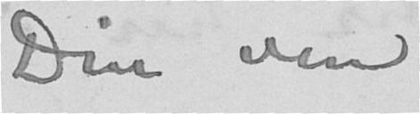Din ven
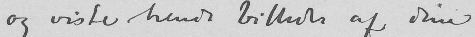og viste hende billeder af dine
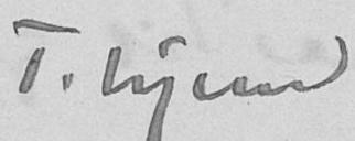T. hjem
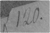120.
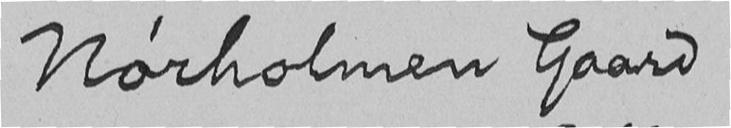Nørholmen Gaard
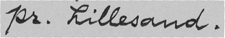pr. Lillesand.
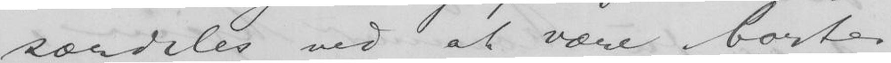særdeles ved at være borte.
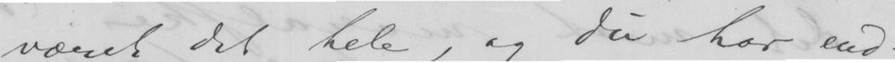været det hele, og Du har end-
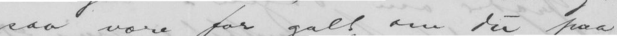saa være for galt om Du paa
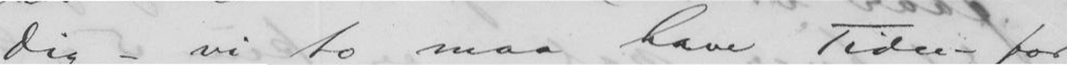Dig - vi to maa have Tiden for
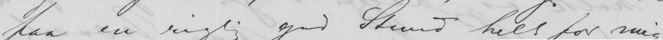faa en rigtig god Stund helt for mig
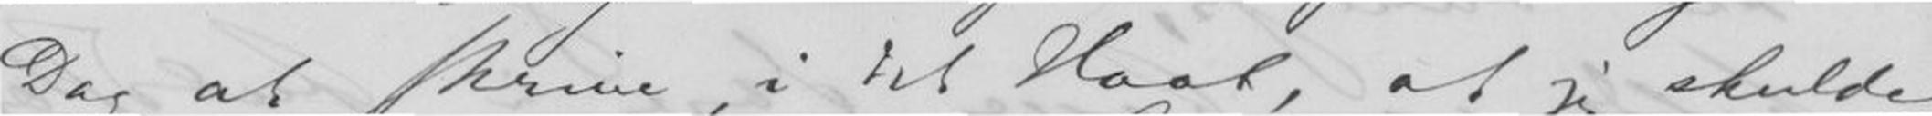Dag at skrive, i det Haab, at jeg skulde
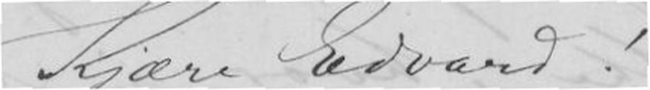Kjære Edvard!
